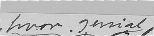hvor genial
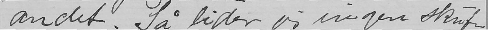andet. Så lider jeg ingen skuf-
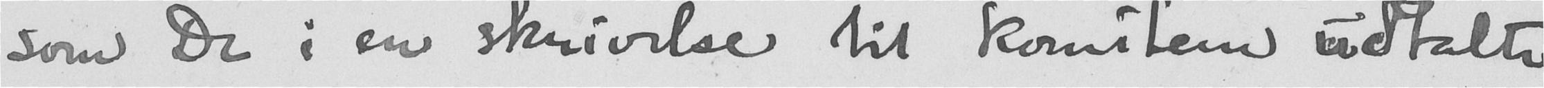som De i en skrivelse til komiteen udtalte
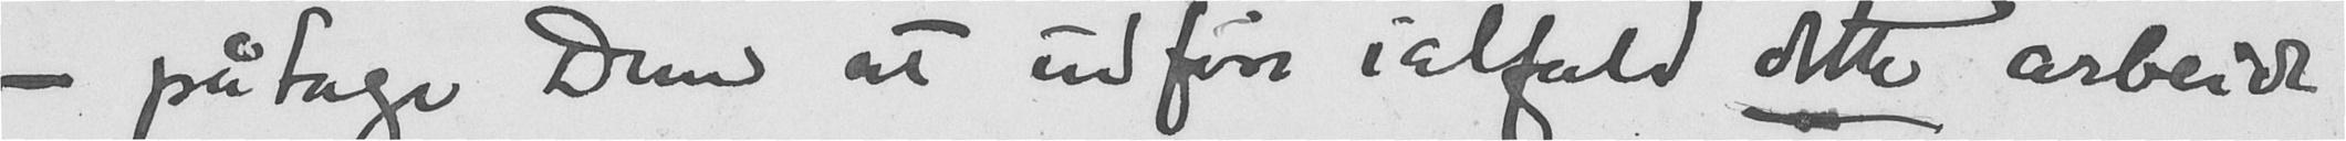- påtage Dem at udføre ialfald dette arbeide.
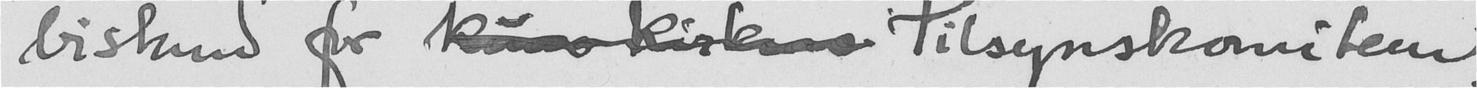bistand for kun kirkens Tilsynskomiteen,
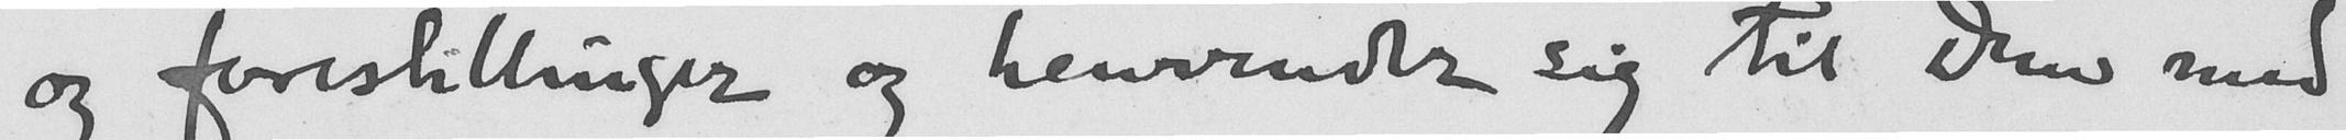og forestillinger og henvender sig til Dem med
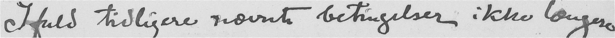Ifald tidligere nævnte betingelser ikke længere
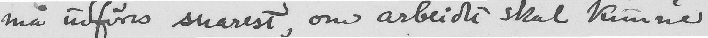må udføres snarest, om arbeidet skal kunne
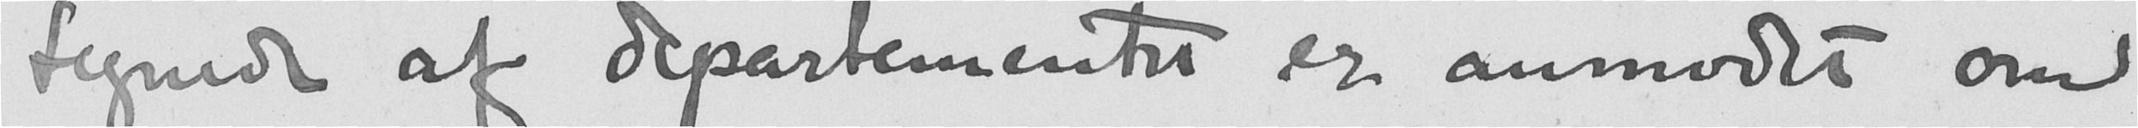tegnede af departementet er anmodet om
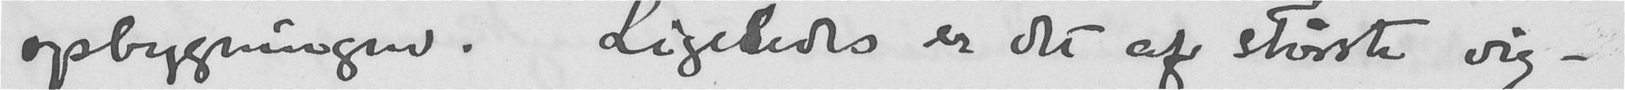opbygningen. Ligeledes er det af største vig-
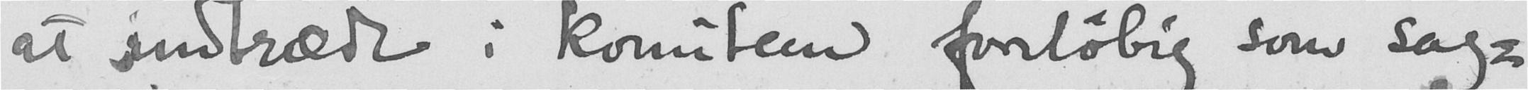at indtræde i komiteen foreløbig som sag-
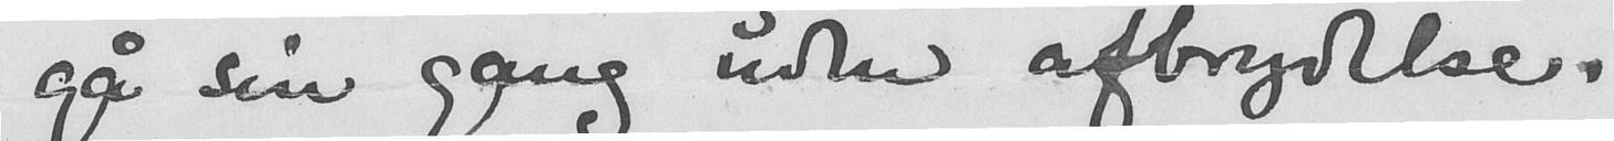gå sin gang uden afbrydelse.
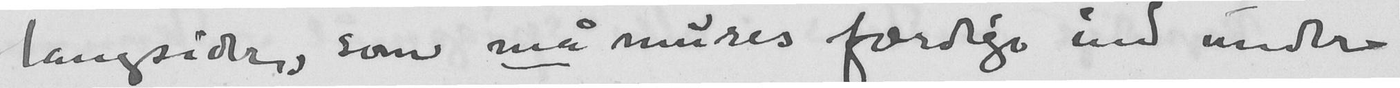langsider, som må mures færdige ind under
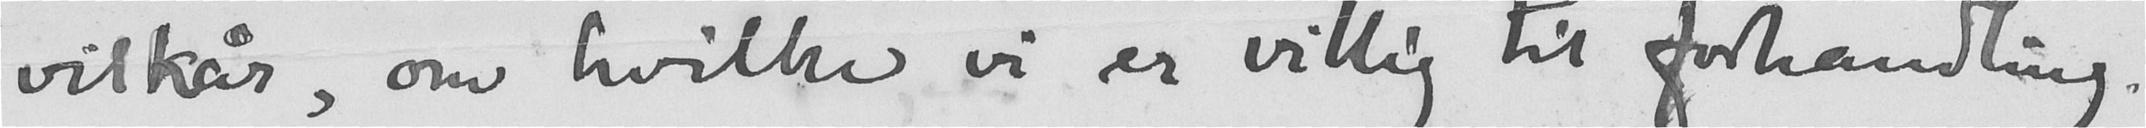vilkår, om hvilke vi er villig til forhandling.
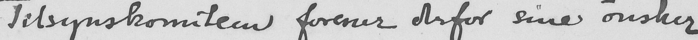Tilsynskomiteen forener derfor sine ønsker
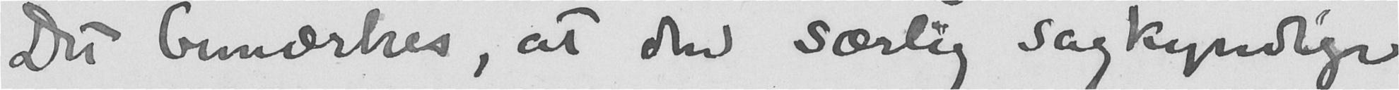Det bemærkes, at den særlig sagkyndige
